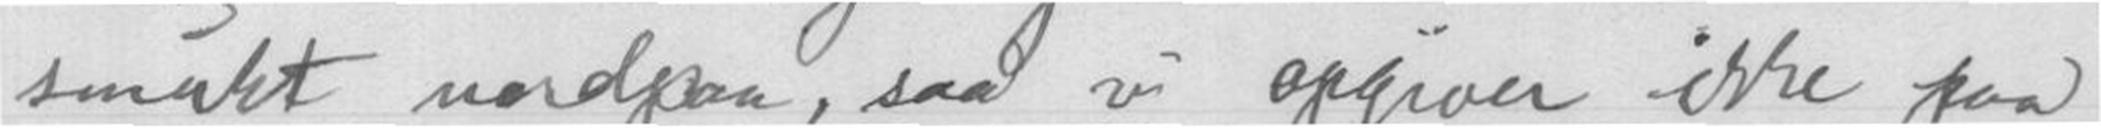smukt nordpaa, saa vi opgiver ikke paa
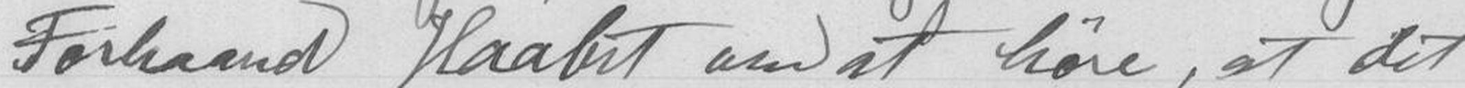Forhaand Haabet om at høre, at det
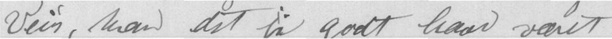Veir, kan det jo godt have været
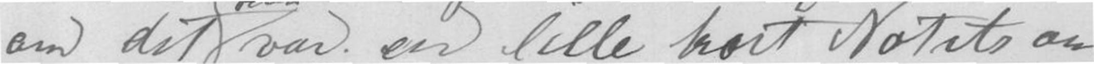om det var en lille kort Notis om
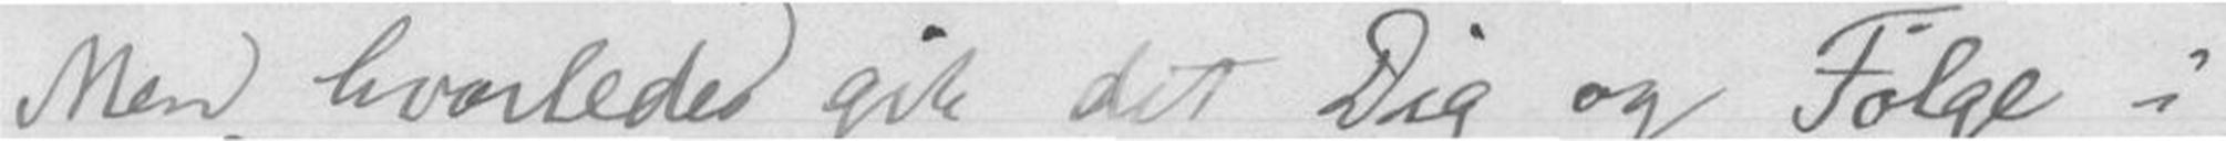Men hvorledes gik det Dig og Følge i
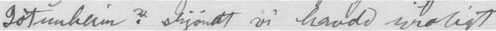Jotunheimen? skjøndt vi havde uroligt
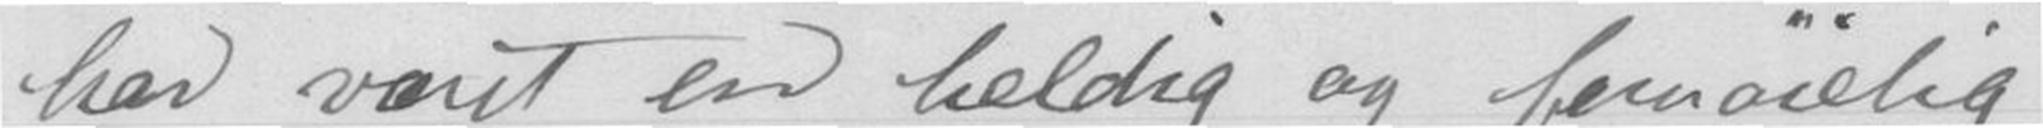har været en heldig og fornøielig
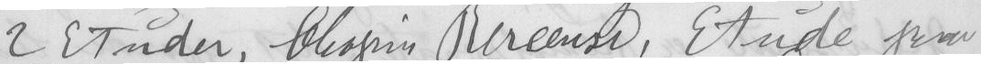2 Etuder, Chopin Bercense, Etude paa
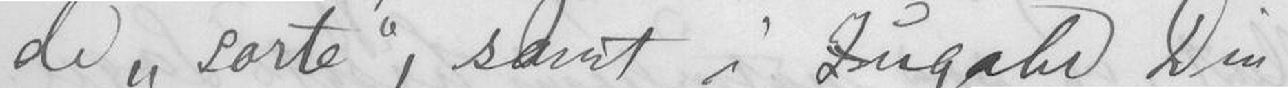de sorte, samt i Zugabe Din
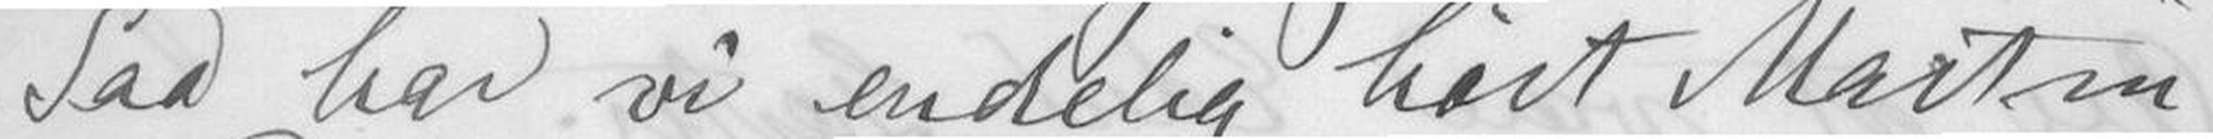Saa har vi endelig hørt Martin
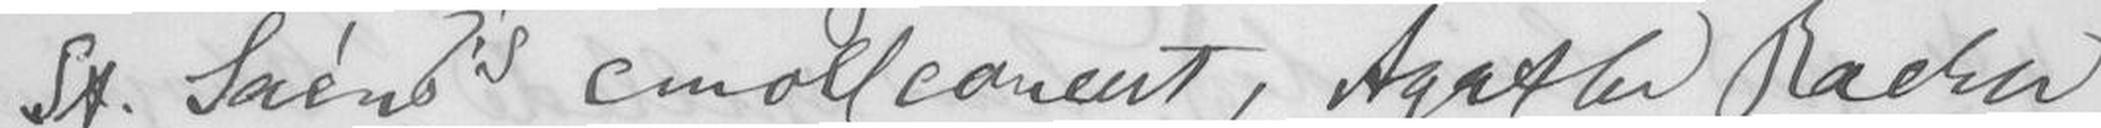St. Saens's cmollconcert, Agathe Backer
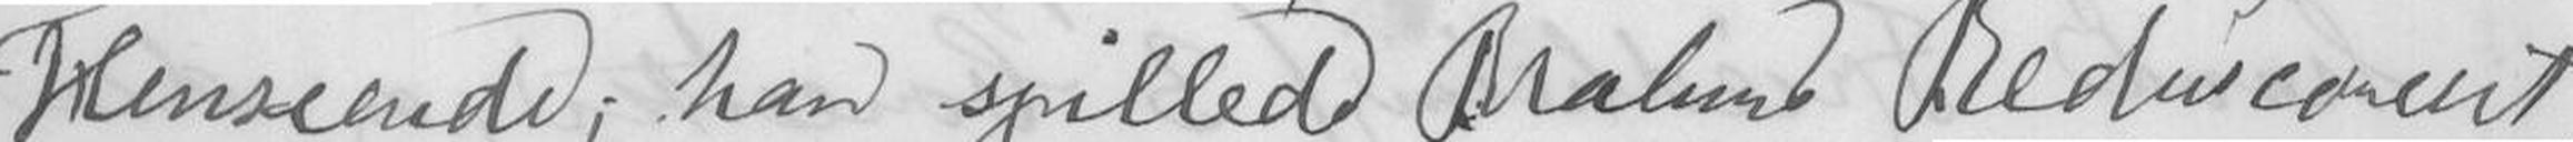Henseende; han spillede Brahms Bedurconcert,
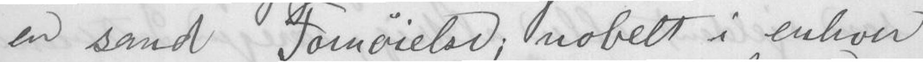en sand Fornøielse; nobelt i enhver
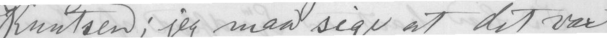Knutsen; jeg maa sige at det var
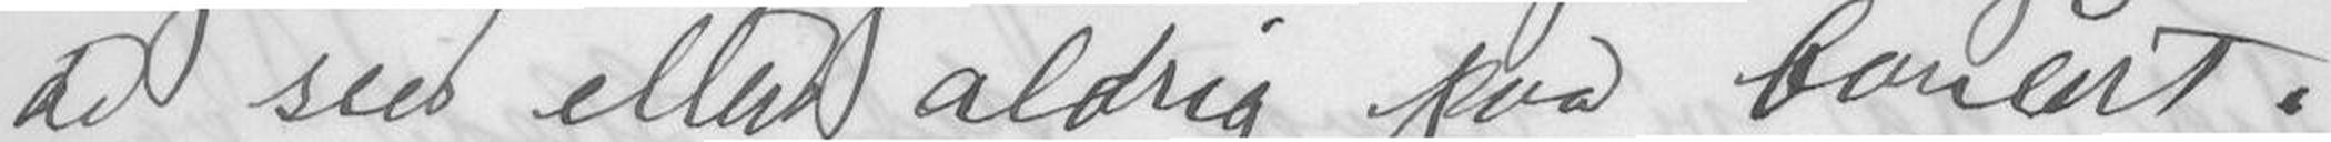de sees ellers aldrig paa Concert.
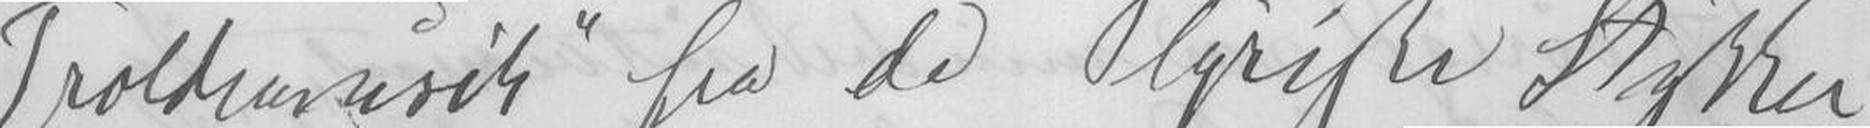Troldmusik fra de lyriske Stykker.
